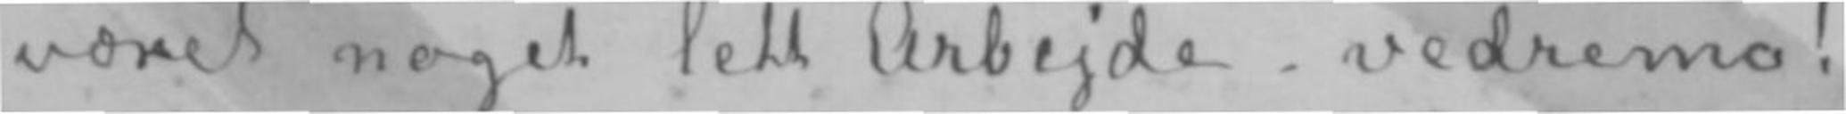været noget lett Arbejde - vedremo!
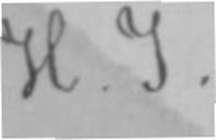H. I.
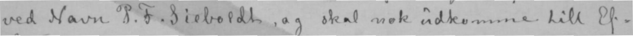ved Navn P. F. Sieboldt, og skal nok udkomme till Ef-
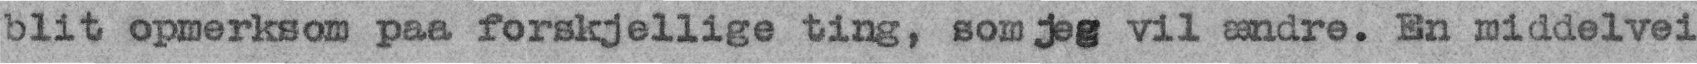blit opmerksom paa forskjellige ting, som jeg vil ændre. En middelvei
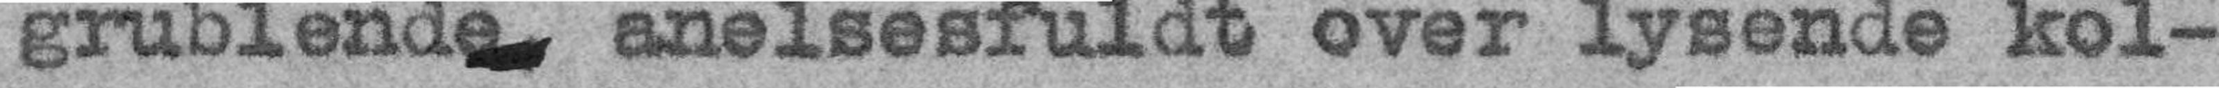grublende anelsesfuldt over lysende kol-
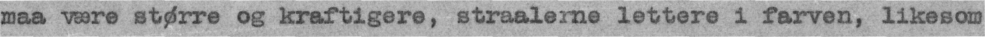maa være større og kraftigere, straalerne lettere i farven, likesom
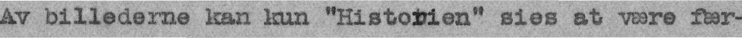Av billederne kan kun «Historien» sies at være fær-
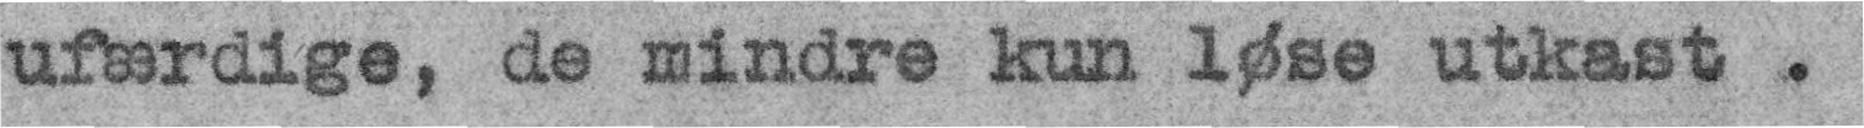ufærdige, de mindre kun løse utkast.
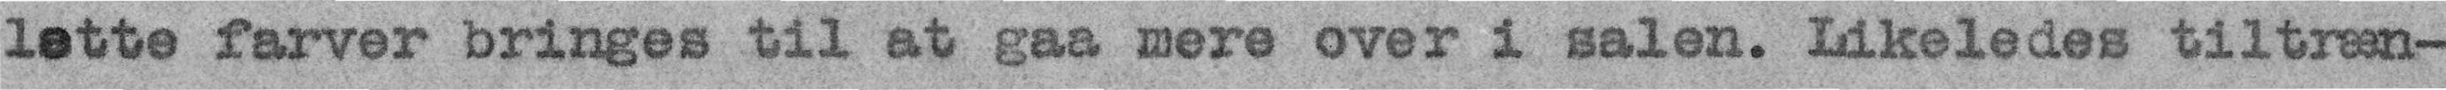lette farver bringes til at gaa mere over i salen. Likeledes tiltræn-
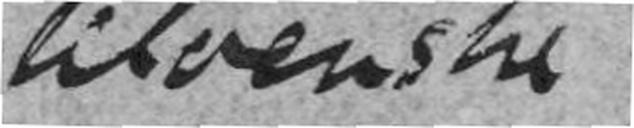tilvenstre
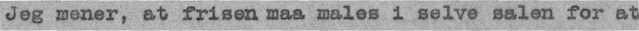Jeg mener, at frisen maa males i selve salen for at
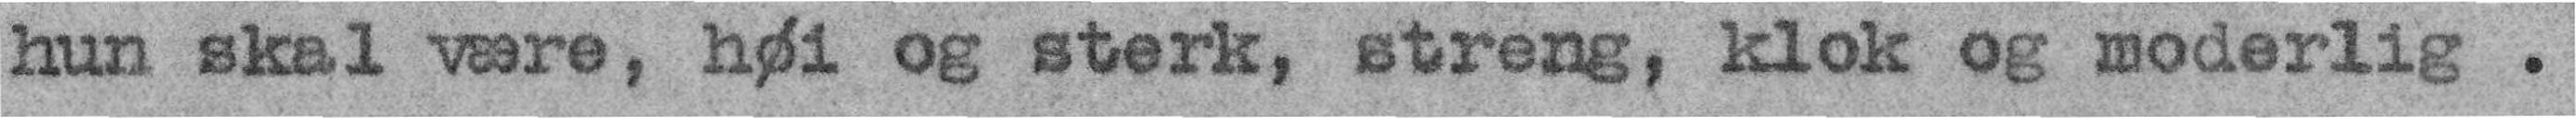hun skal være, høi og sterk, streng, klok og moderlig.
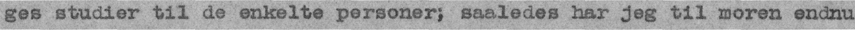ges studier til de enkelte personer; saaledes har jeg til moren endnu
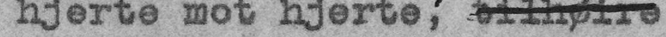hjerte mot hjerte; tilhøire
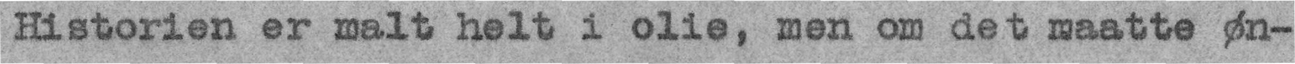Historien er malt helt i olie, men om det maatte øn-
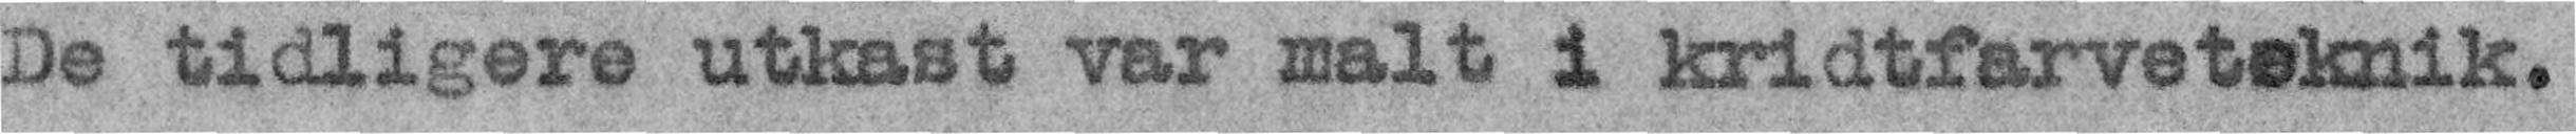De tidligere utkast var malt i kridtfarveteknik.
